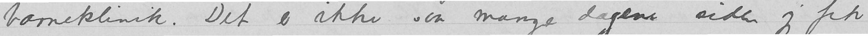barneklinik. Det er ikke saa mange dagene siden jeg fik
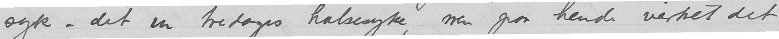syk – det var  tredages halsesyke, men paa hende virket det
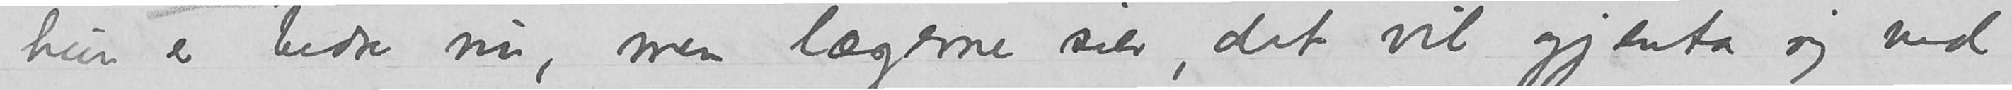– hun er bedre nu, men lægerne sier, det vil gjenta sig ved
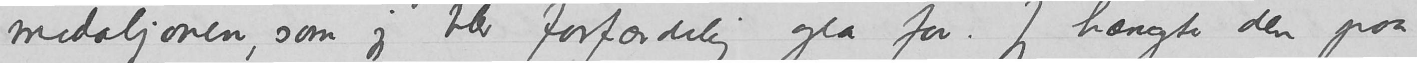medaljonen, som jeg blev forfærdelig gla for. Jeg hængte den paa
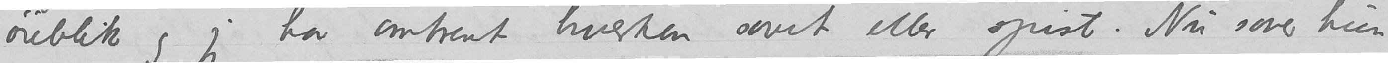øieblik og jeg har omtrent hverken sovet eller spist. Nu sover hun
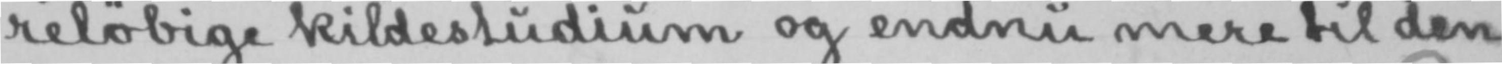reløbige kildestudium og endnu mere til den
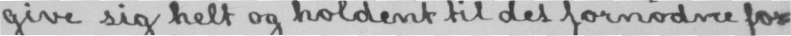give sig helt og holdent til det fornødne fo-
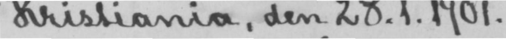Kristiania, den 28.1.1901.
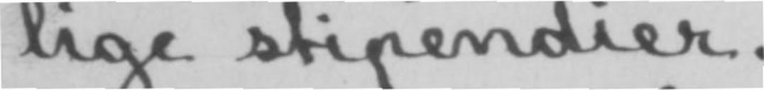lige stipendier.
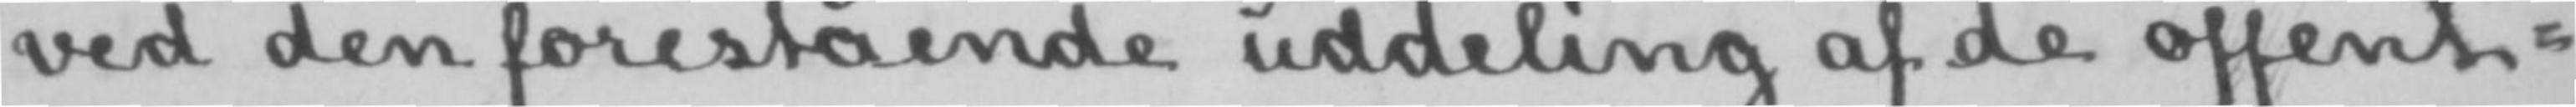ved den forestående uddeling af de offent-
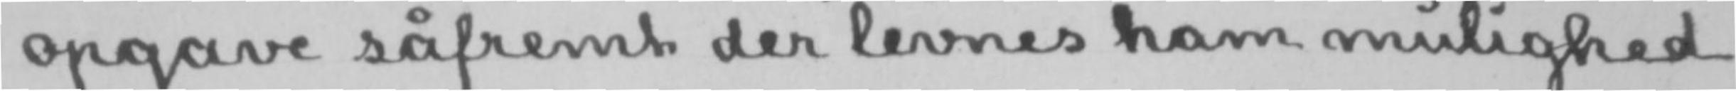opgave såfremt der levnes ham mulighed
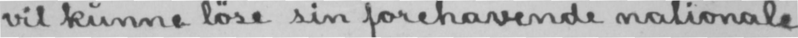vil kunne løse sin forehavende nationale
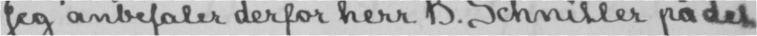Jeg anbefaler derfor herr B. Schnitler på det
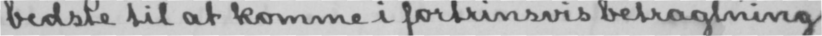bedste til at komme i fortrinsvis betragtning
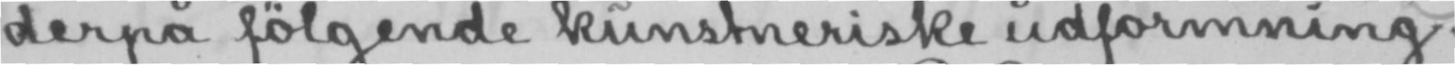derpå følgende kunstneriske udformning.
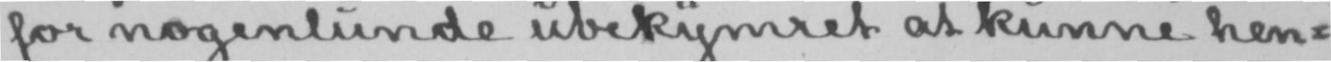for nogenlunde ubekymret at kunne hen-
